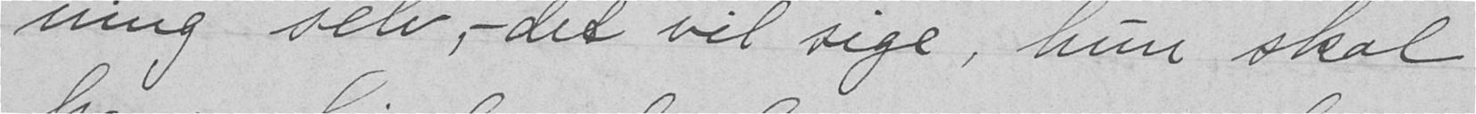ning selv, - det vil sige, hun skal
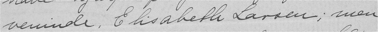veninde, Elisabeth Larsen; men
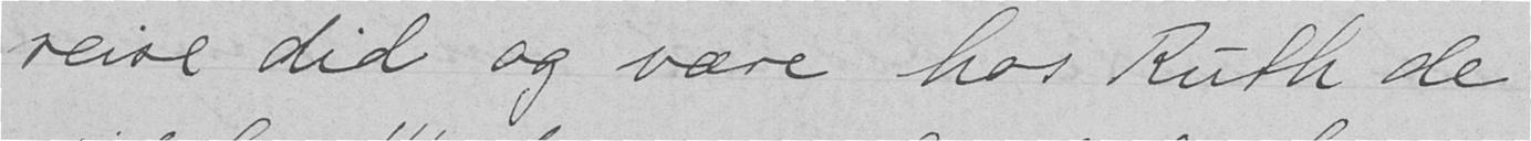reise did og være hos Ruth de
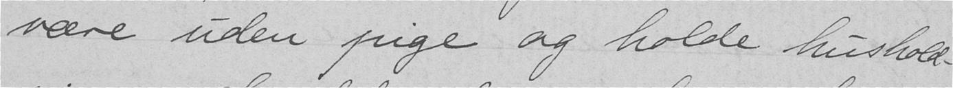være uden pige og holde hushold-
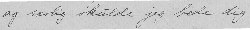og særlig skulde jeg bede dig
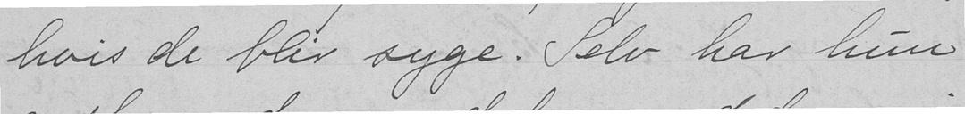hvis de blir syge. Selv har hun
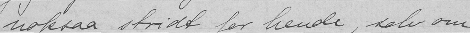noksaa stridt for hende, selv om
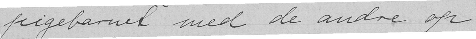pigebarnet med de andre op
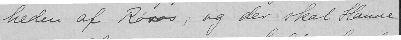heden af Røros; og der skal Hanne
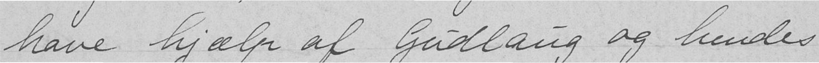have hjælp af Gudlaug og hendes
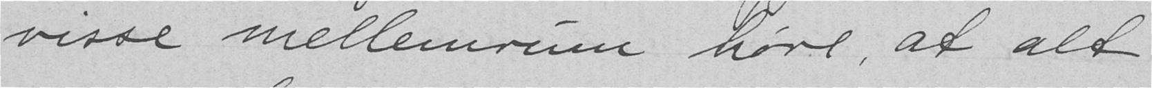visse mellemrum høre, at alt
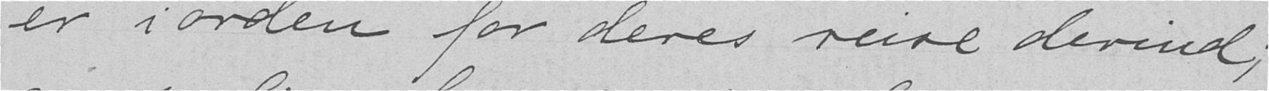er i orden for deres reise derind;
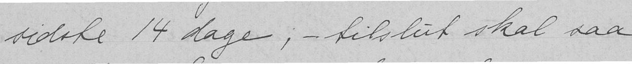sidste 14 dage, - tilslut skal saa
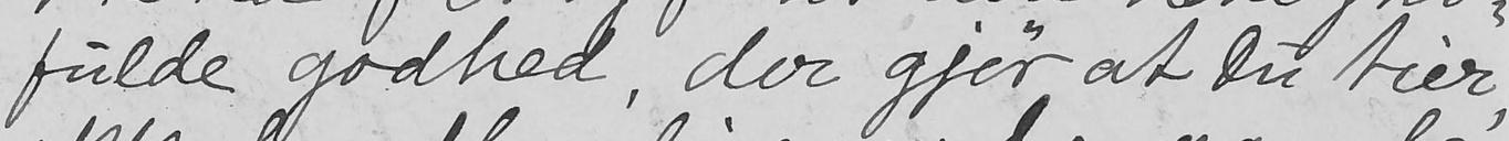fulde godhed, der gjør at Du tier,
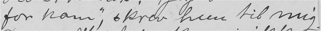for ham" skrev hun til mig.
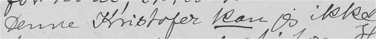Denne Kristofer kan jeg ikke
